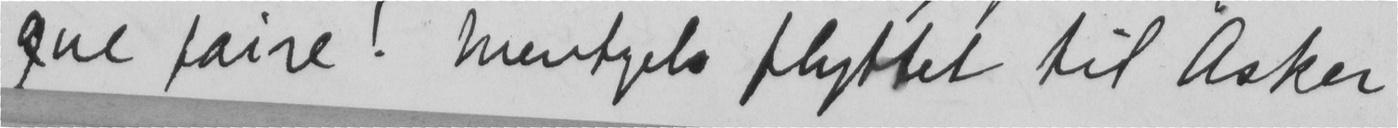que faire! Wentzels flyttet til Asker
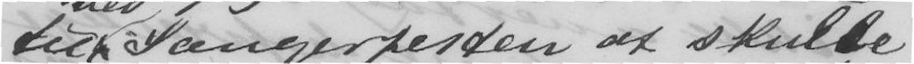til ved Sangerfesten at skulle
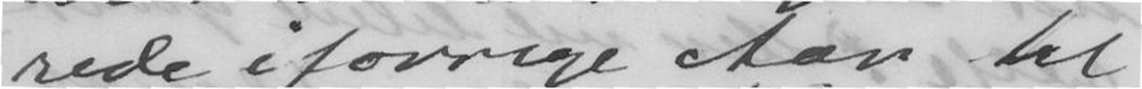rede i forrige Aar til
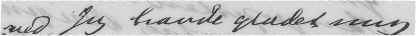Jeg havde glædet mig
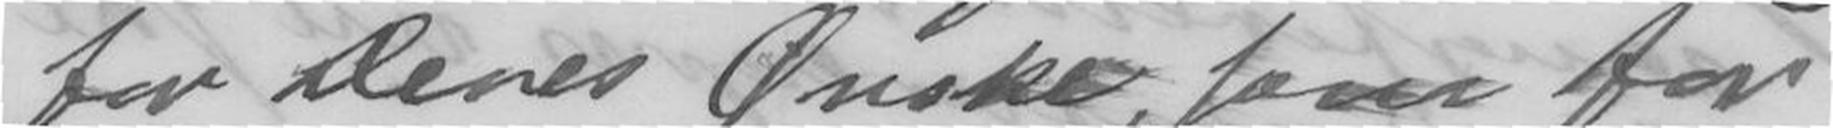for Deres Ønske, som for
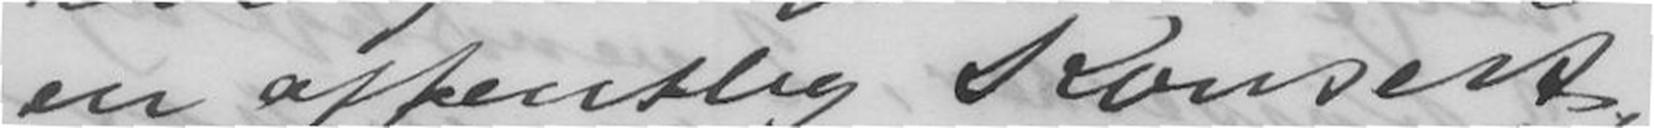en offentlig Konsert
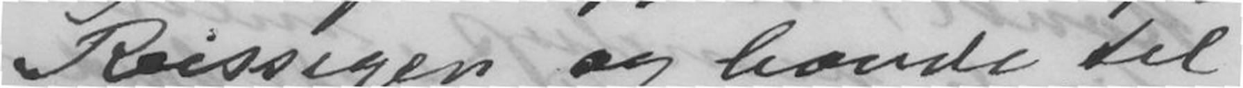Reissiger og havde til
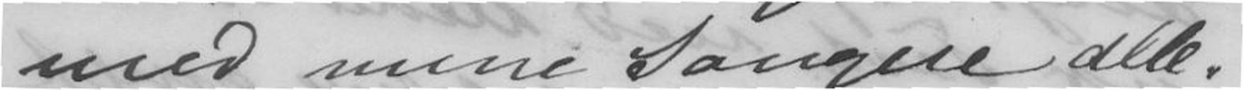med mine Sangere alle-
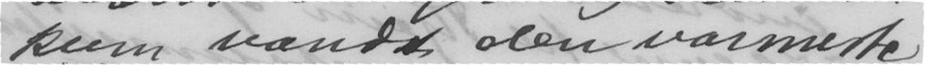kum vandt den varmeste
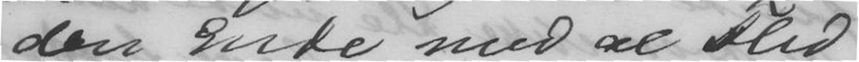den Ende med al Flid
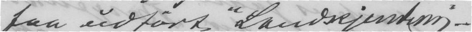faa udført "Landskjending"
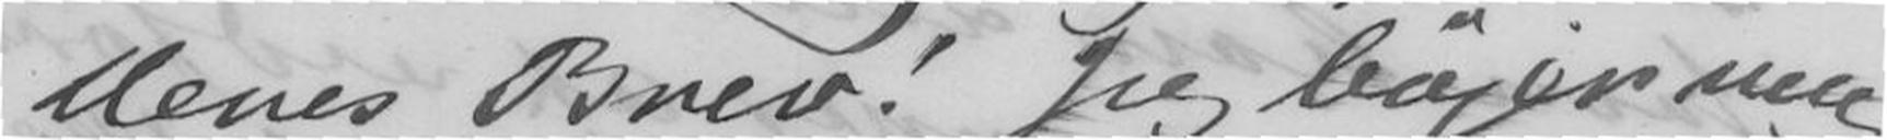Deres Brev! Jeg bøjer mig
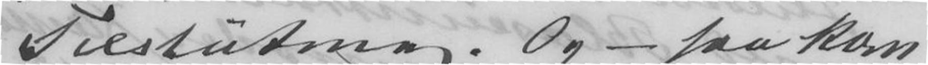Tilslutning. Og - saa kom
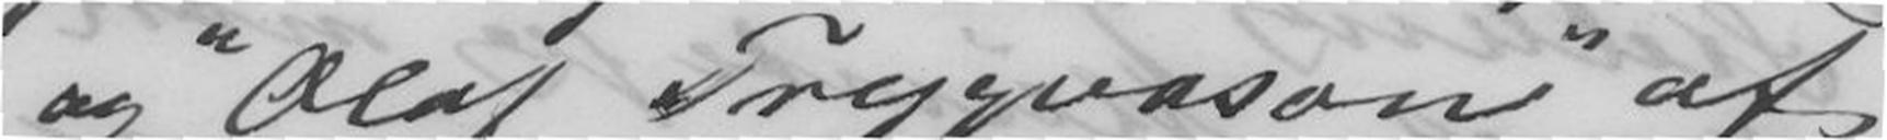og "Olaf Trygvason" af
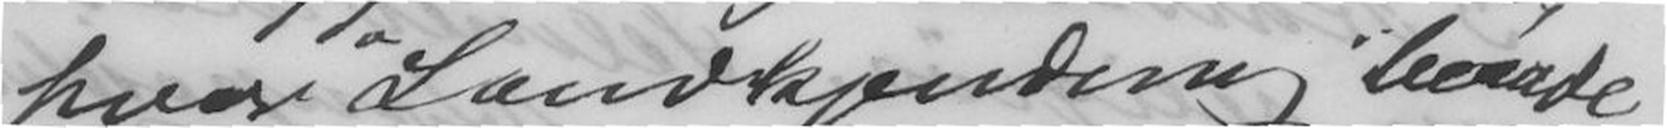hvor "Landkjending" baade
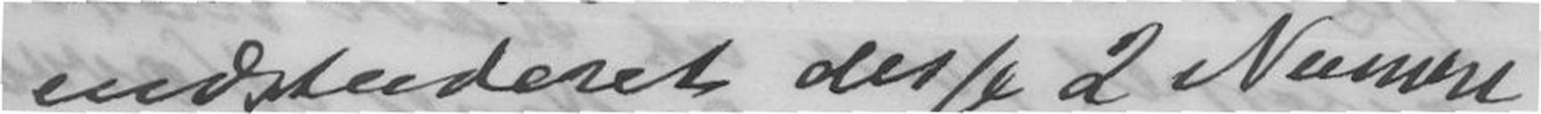indstuderet disse 2 Numere
